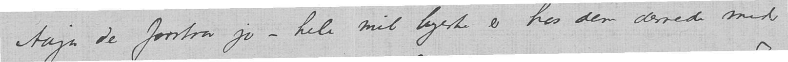Aaja De forstaar jo - hele mit hjerte er hos dem dernede med
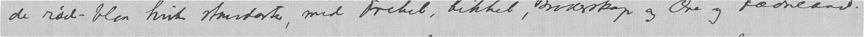de rød-blaa hvite standarter, med Frihet, Likhet, Broderskap og Ære og Fædreland.
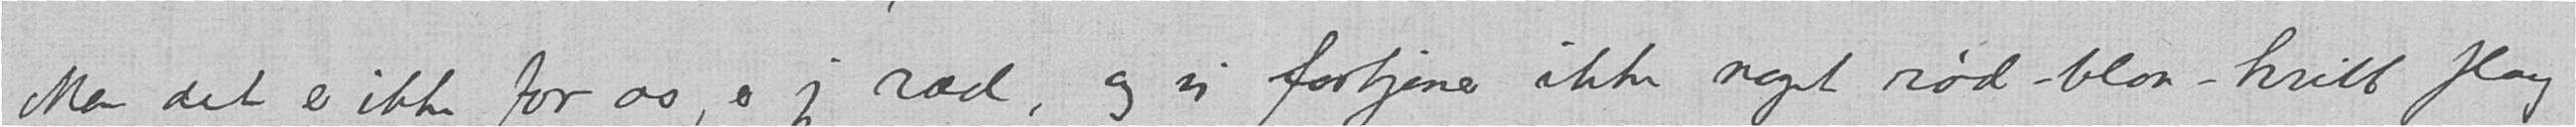Men det er ikke for os, er jeg ræd, og vi fortjener ikke noget rød-blaa-hvitt flag
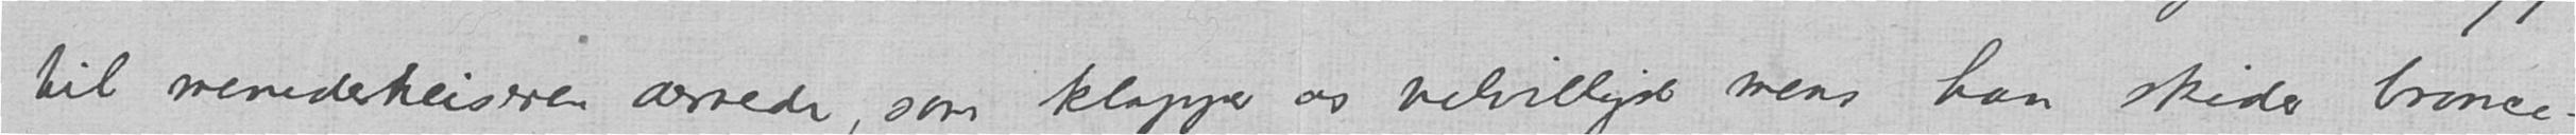til menederkeiseren dernede, som klapper os velvilligst mens han skider bronce-
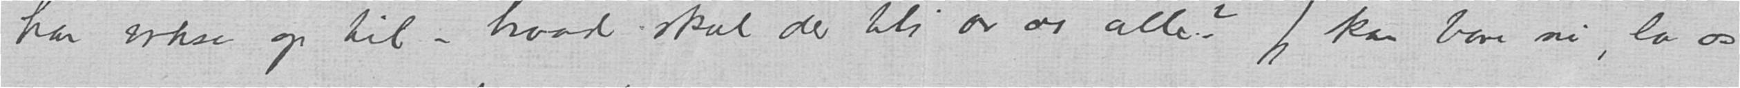han vokse op til – hvad skal der bli av os alle? Jeg kan bare si, la os
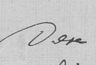Deres
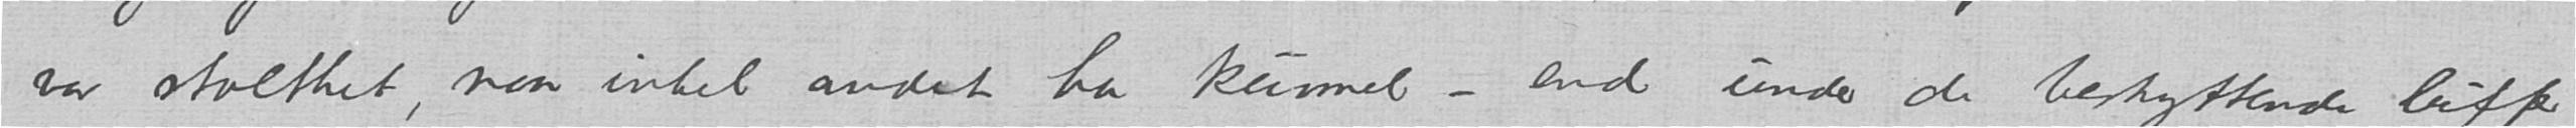vor stolthet, naar intet andet har kunnet - end under de beskyttende luffer
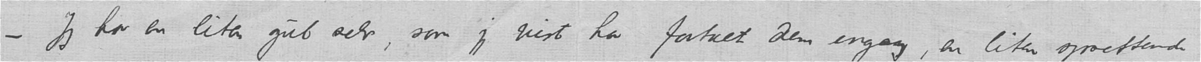Jeg har en liten gut selv, som jeg vist har fortalt dem engang, en liten sprettende
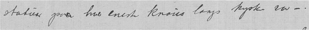statuer paa hver eneste knaus langs kysten vor -.
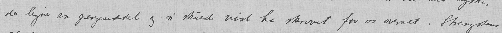der ligner en pengeseddel og vi skulde vist ha skrevet for os overalt. Strengstens
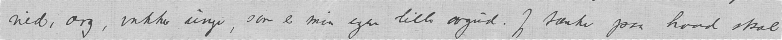nid, arg, vakker unge, som er min egen lille avgud. Jeg tænke paa hvad skal
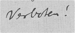Verboten!
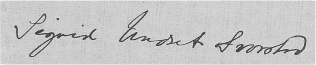Sigrid Undset Svarstad
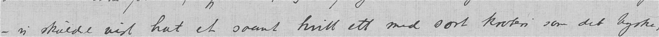- vi skulde vist hat et saant hvitt ett med sort kroteri som det tyske,
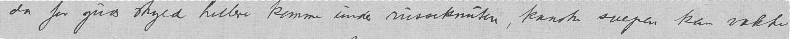da for guds skyld hellere komme under russeknuten, kanske svepen kan vække
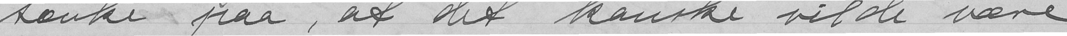tænke paa, at det kanske vilde være
